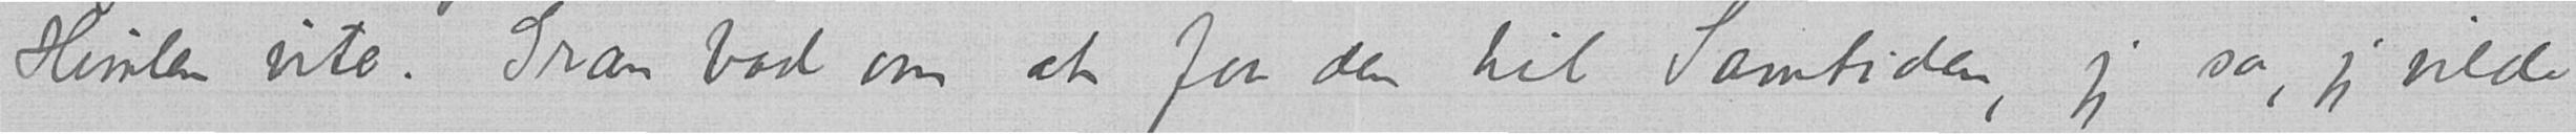Himlen vite. Gran bad om at faa den til Samtiden, jeg sa, jeg vilde
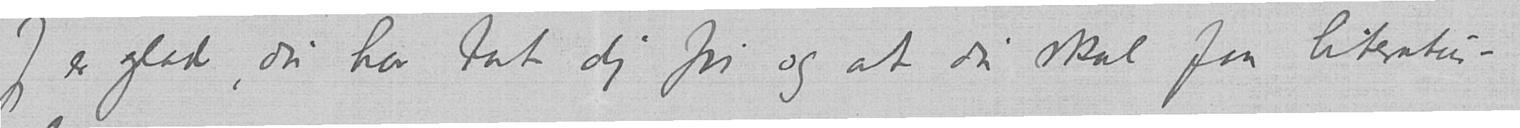Jeg er glad, du har tat dig fri og at du skal faa literatur-
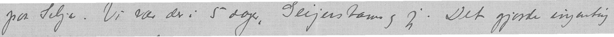paa Selja. Vi var der i 5 dager, Geijerstams og jeg. Det gjorde ingenting
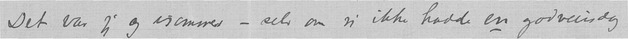Det var jeg og isommer – selv om vi ikke hadde en godveirsdag
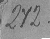272.
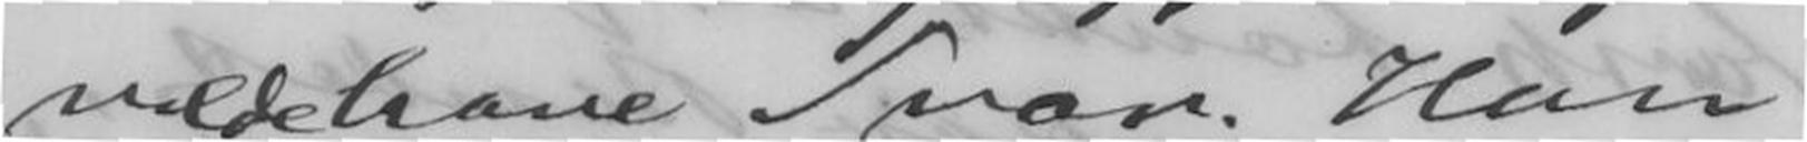vilde have Svar. Han
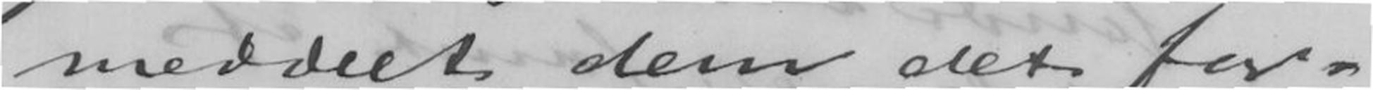meddelt dem det for-
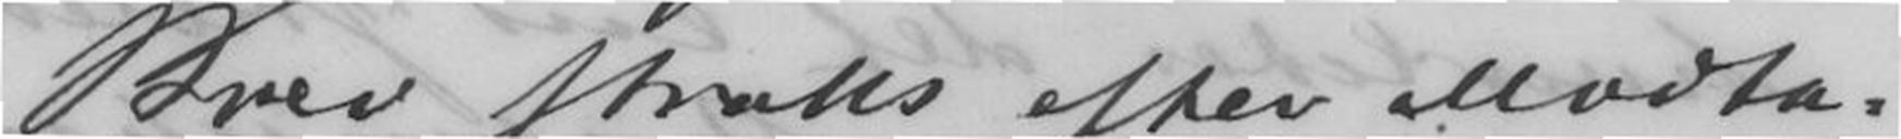Brev straks efter Mota-
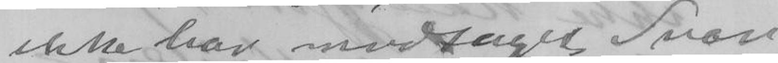ikke har modtaget Svar,
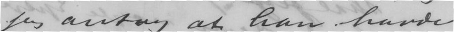jeg antog at han havde
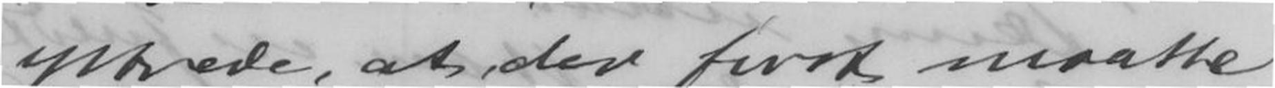yttrede, at der først maatte
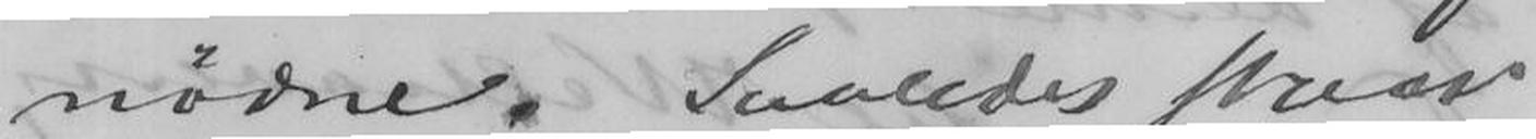nødne. Saaledes staar
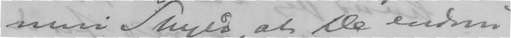min Skyld, at De endnu
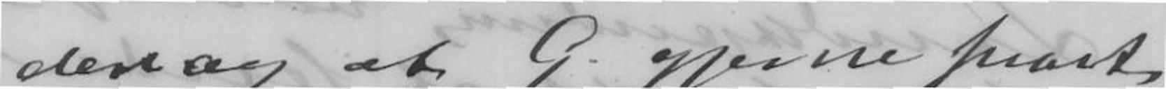der og at G. gjerne snart
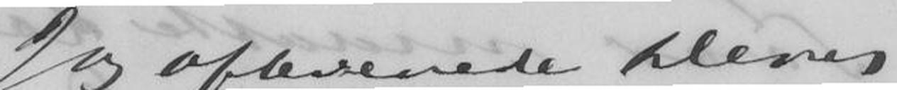Jeg afleverede Deres
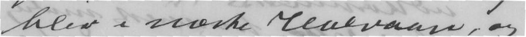blev i næste Halvaar, og
